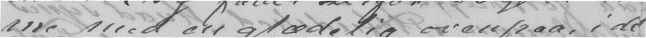me med en glædelig ovenpaa, i det
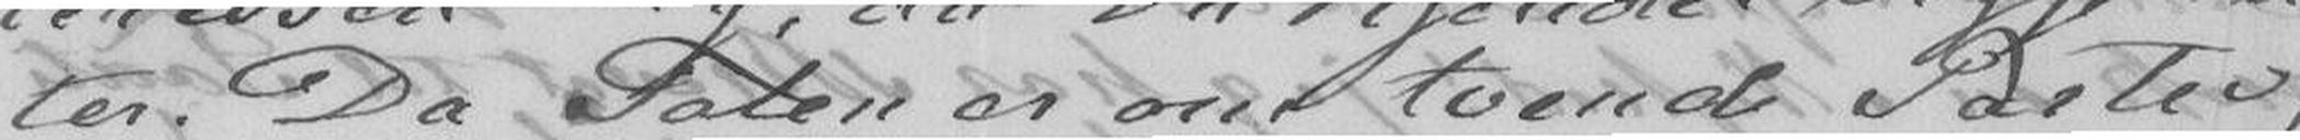ter. Da Talen er om tvende Parter,
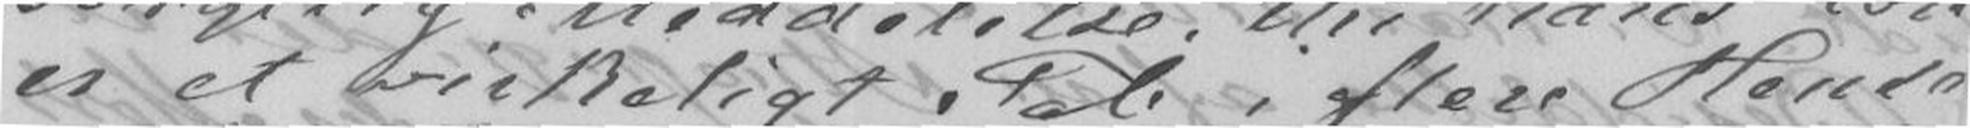er et virkeligt Tab i flere Hende-
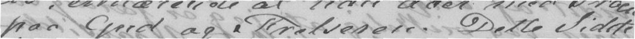paa Gud og Frelseren. Dette Sidste
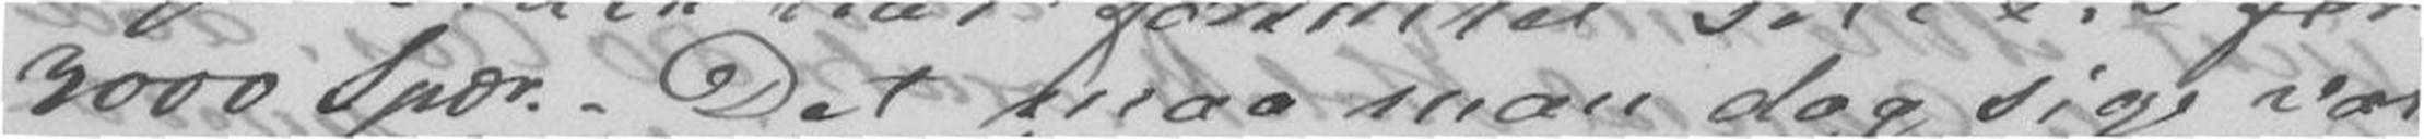3000 Spdr. - Det maa man dog sige var
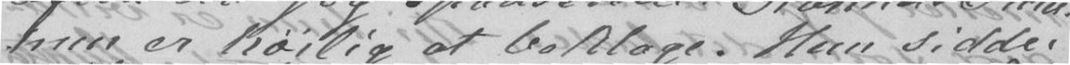hun er høilig at beklage. Hun sidder
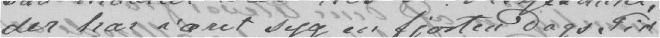der har været syg en fjorten Dags Tid
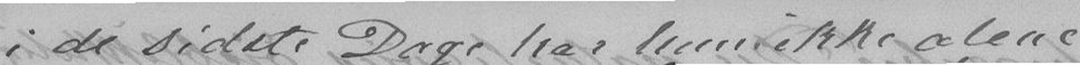i de sidste Dage har hun ikke alene
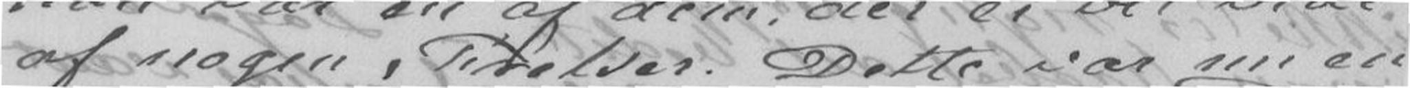af nogen Frelser. Dette var nu en
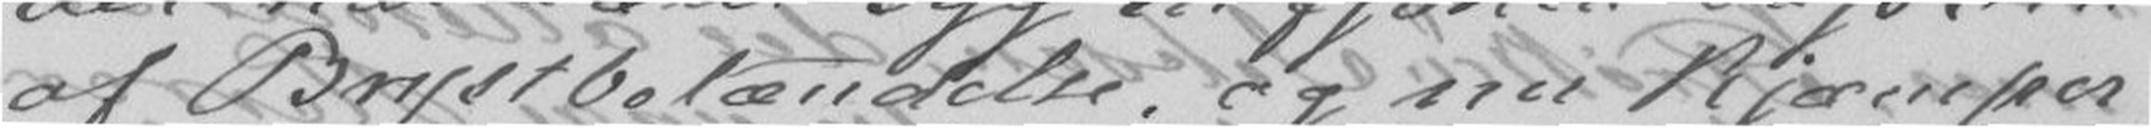af Brystbetændelse, og nu kjæmper
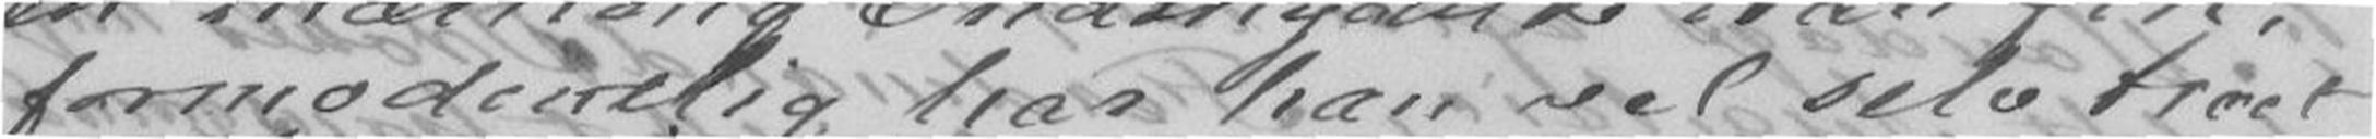formodentlig har han vel selv troet
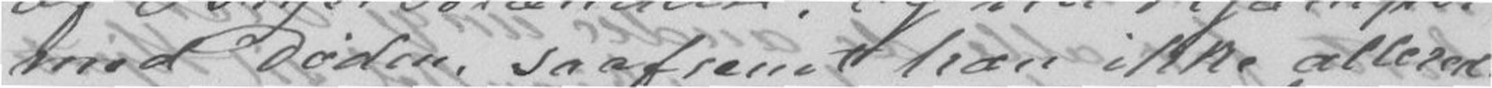med Døden, saafremt han ikke allerede
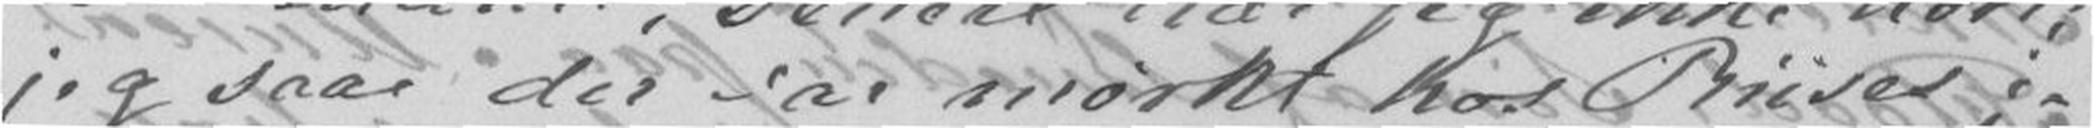jeg saae der var mørkt hos Riises i-
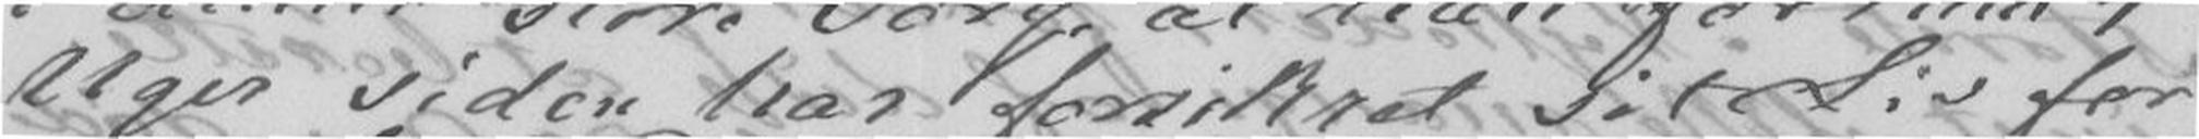Uger siden har forsikret sit Liv for
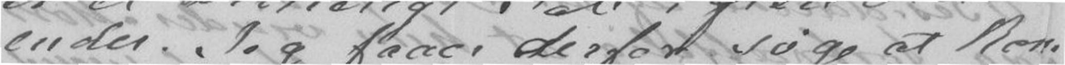ender. Jeg faaer derfor søge at kom-
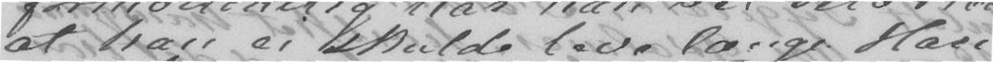at han ei skulde leve længe. Han
